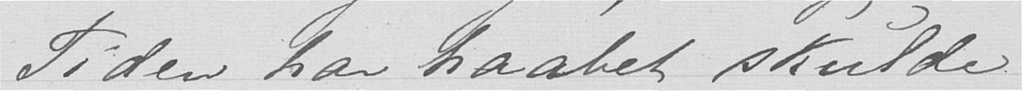Tiden har haabet skulde
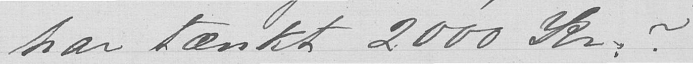har tænkt 2000 Kr.?
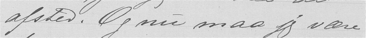afsted. Og nu maa jeg være
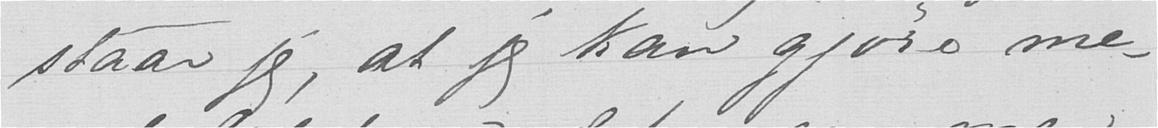staar jeg, at jeg kan gjøre me-
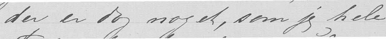der er dog noget, som jeg hele
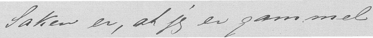Saken er, at jeg er gammel
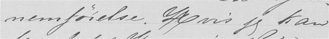nemførelse. Hvis jeg kan
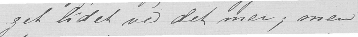get lidet ved det mer; men
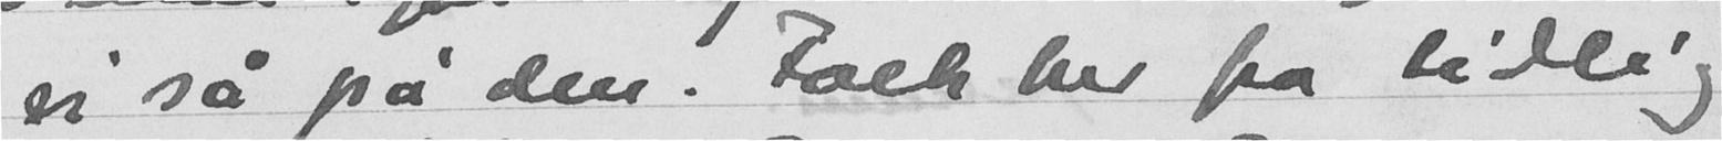vi så pa den. Folk her fra tidlig
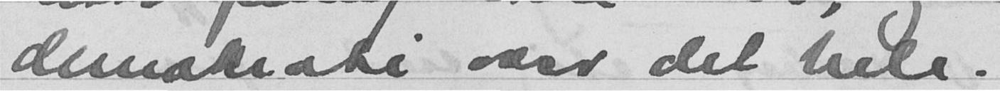demokrati over det hele.
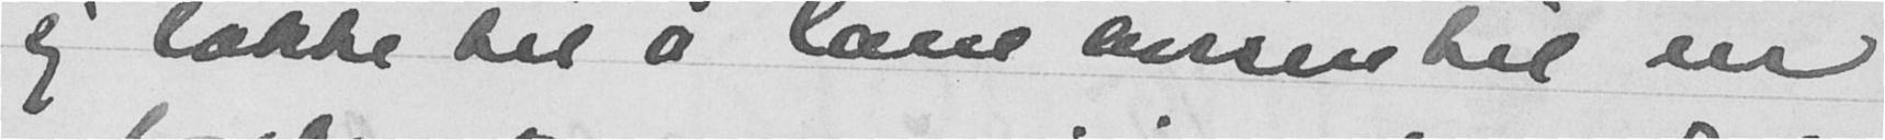sig lokke til å låne avisen til en
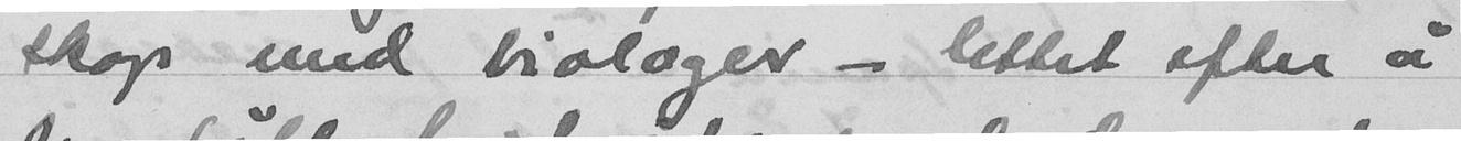skogs med biologer - lettet efter å
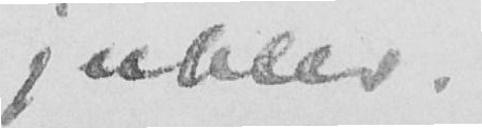jubler.
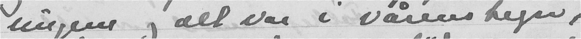ungene og alt var i vårens tegn,
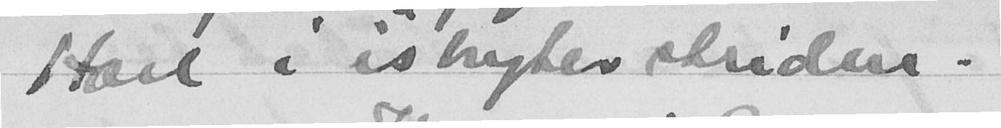Karl i isbryterstriden.
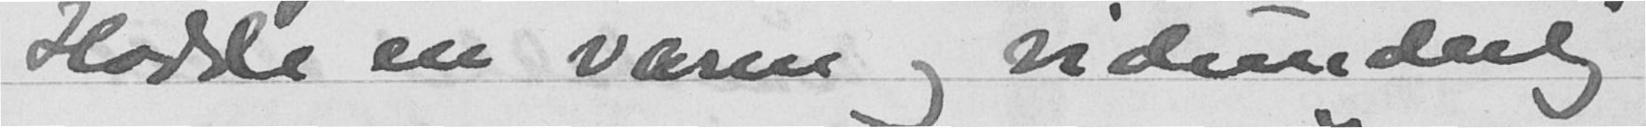Hadde en varm og vidunderlig
